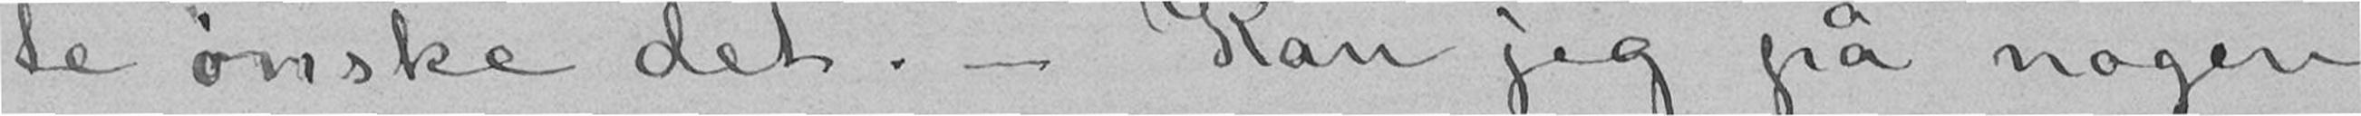te ønske det.- Kan jeg på nogen
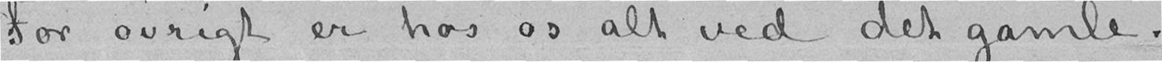For øvrigt er hos os alt ved det gamle.
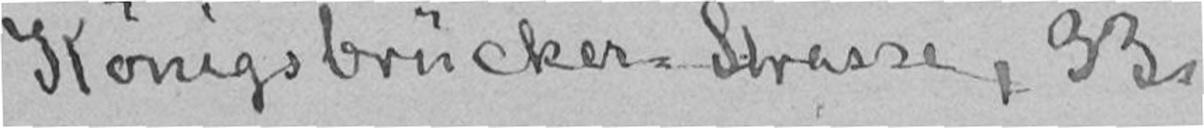Königsbrücker-Strasse, 33.
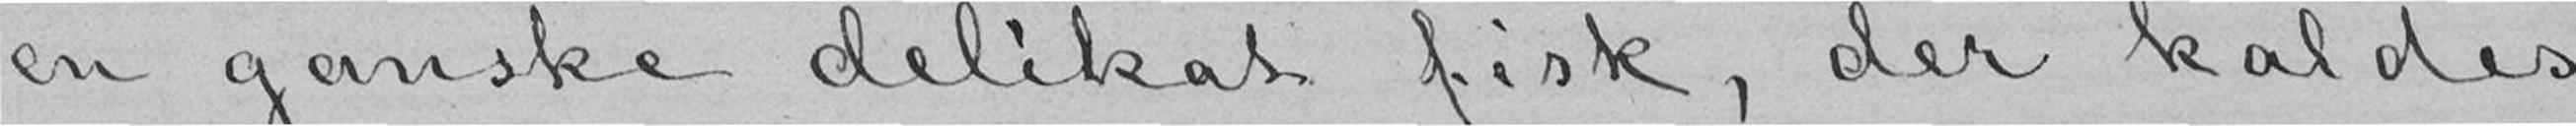en ganske delikat fisk, der kaldes
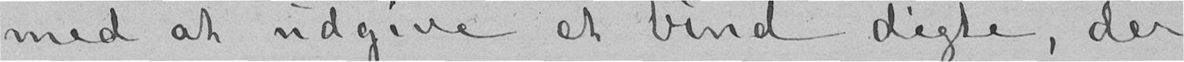med at udgive et bind digte, der
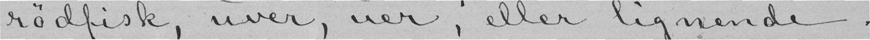rødfisk, uver, uer, eller lignende.
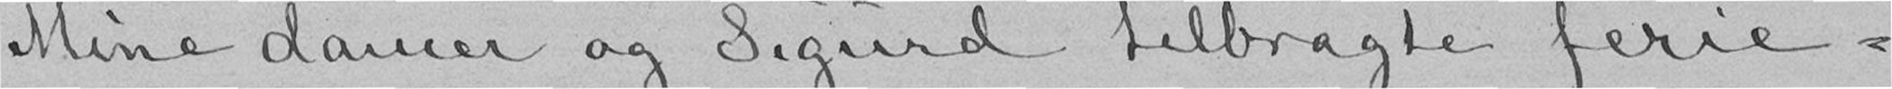Mine damer og Sigurd tilbragte ferie-
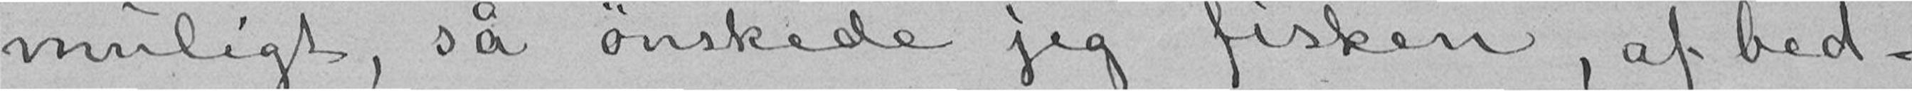muligt, så ønskede jeg fisken, af bed-
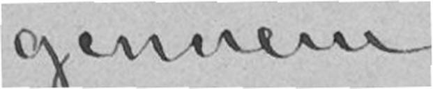gennem
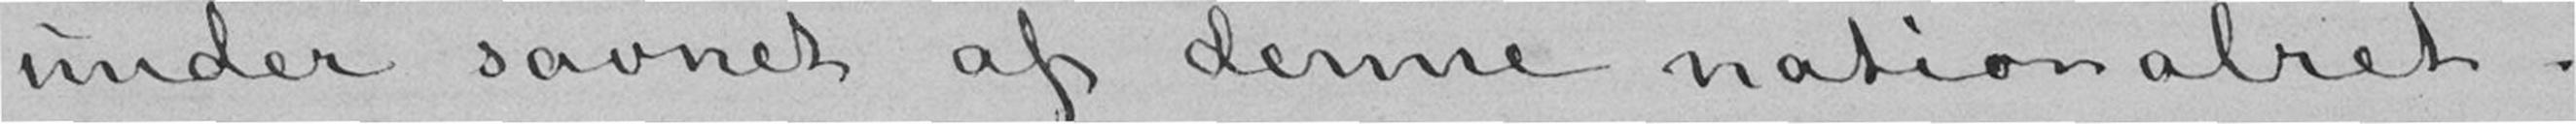under savnet af denne nationalret.
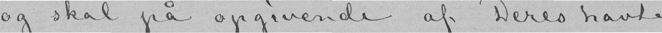og skal på opgivende af Deres havte
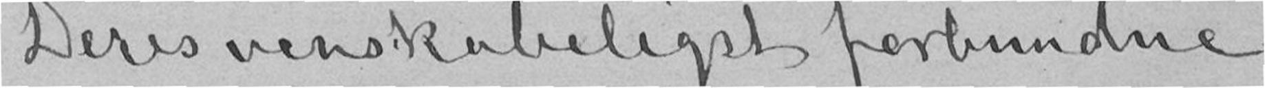Deres venskabeligst forbundne
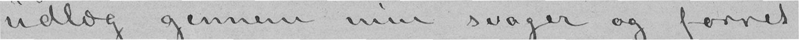udlæg gennem min svoger og forret-
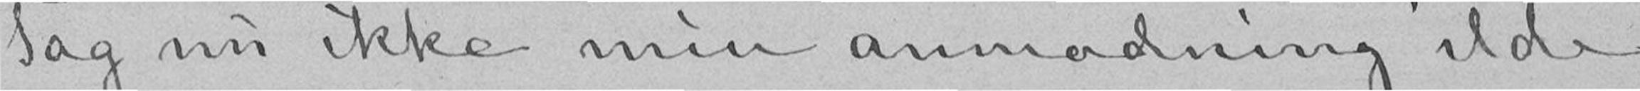Tag nu ikke min anmodning ilde
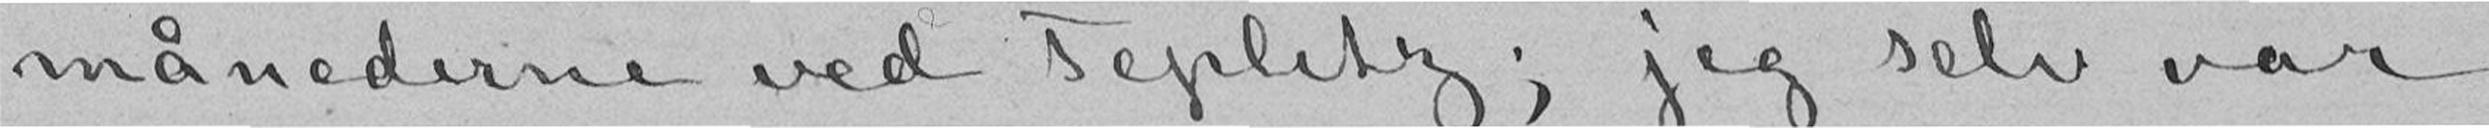månederne ved Teplitz; jeg selv var
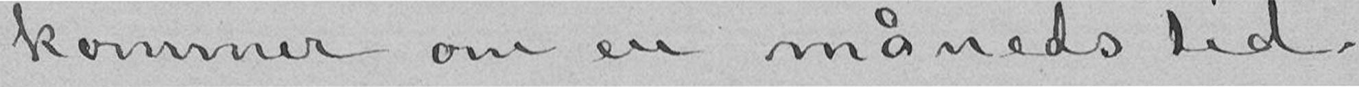kommer om en måneds tid.
